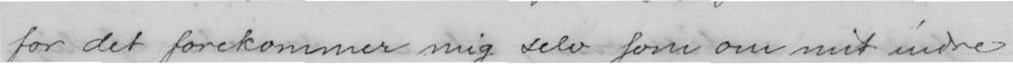for det forekommer mig selv som om mit indre
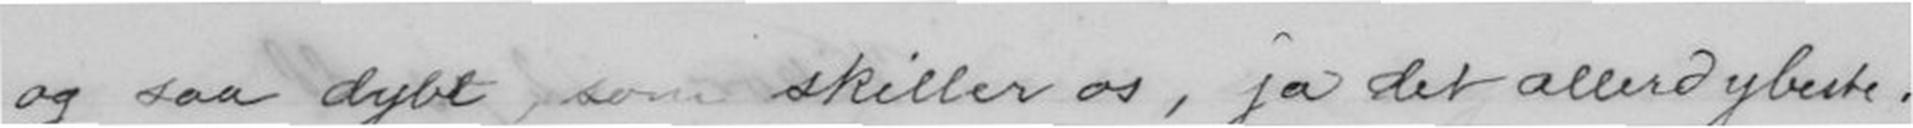og saa dybt, som skiller os, ja det allerdybeste.
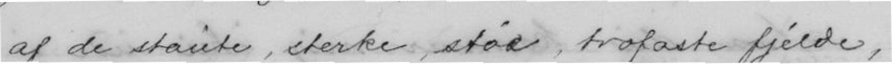af de staute, sterke, støe, trofaste fjelde,
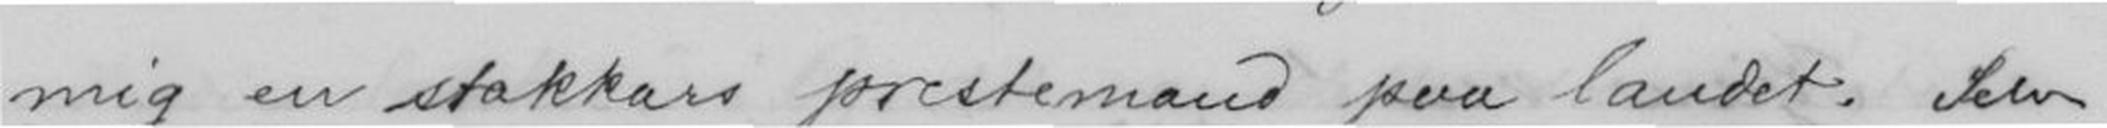mig en stakkars prestemand paa landet. Selv
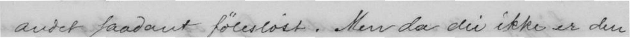andet saadant følesløst. Men da du ikke er den
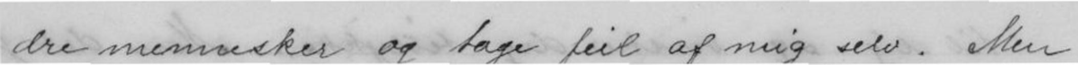dre mennesker og tage feil af mig selv. Men
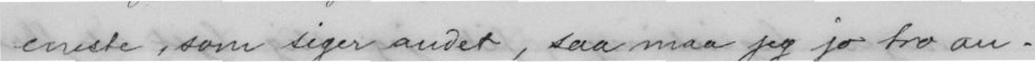eneste, som siger andet, saa maa jeg jo tro an-
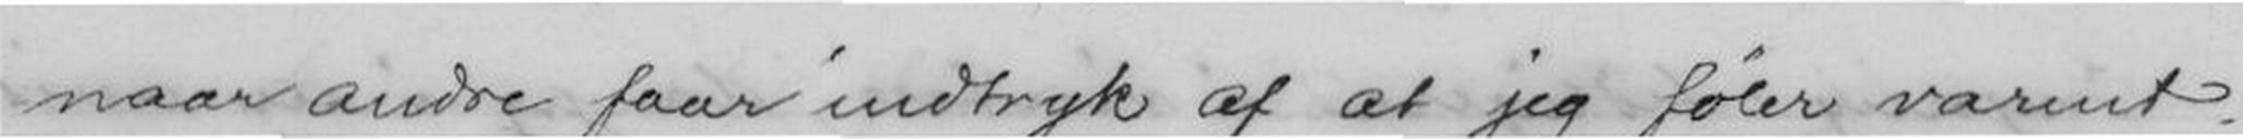naar andre faar indtryk af at jeg føler varmt;
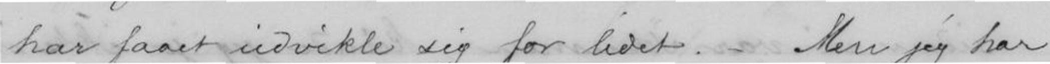har faaet udvikle sig for lidet. Men jeg har
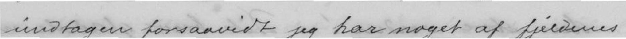undtagen forsaavidt jeg har noget af fjeldernes
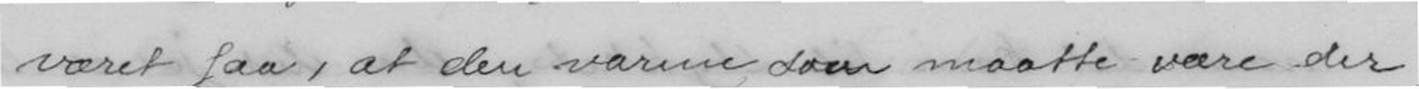været saa, at den varme som maatte være der,
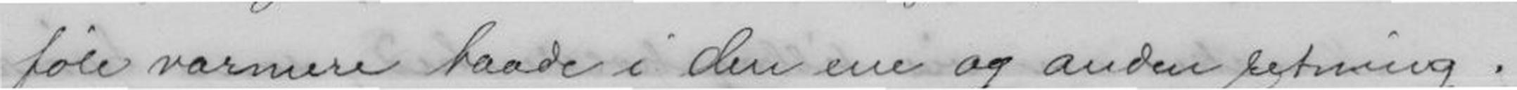føle varmere baade i den ene og den anden retning.
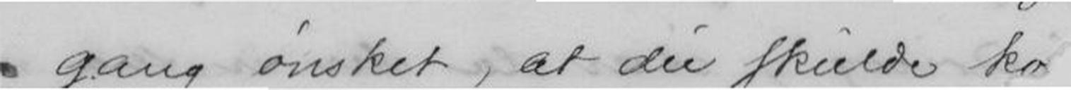gang ønsket, at du skulde ko
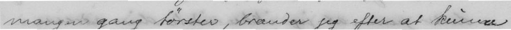mangen gang tørster, brænder jeg efter at kunne
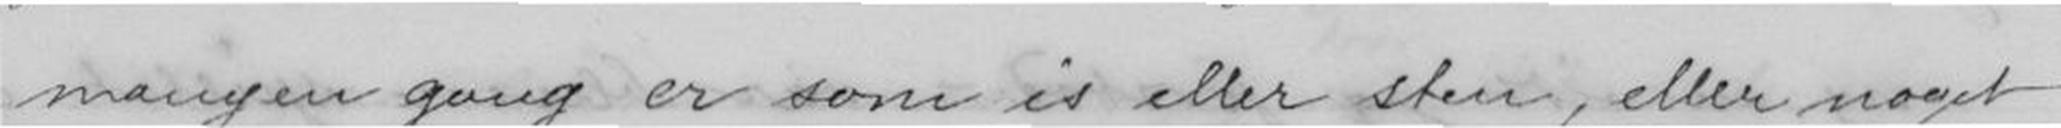mangen gang er som is eller sten, eller noget
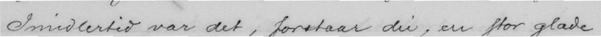Imidlertid var det, forstaar du, en stor glæde
